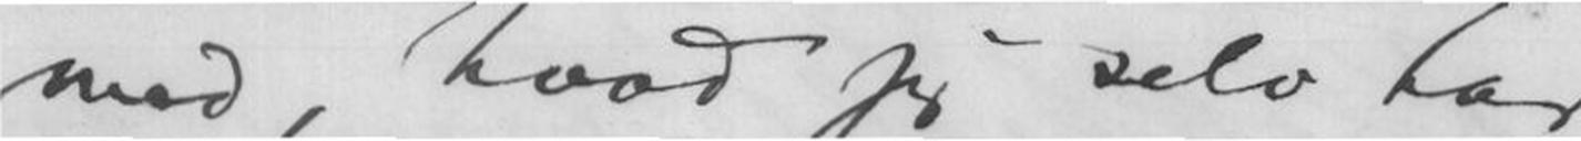med, hvad jeg selv har
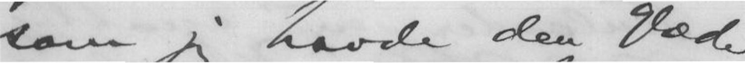som jeg havde den Glæde
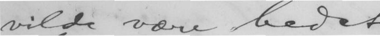vilde være bedst
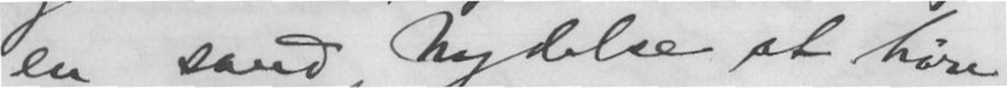en sand Nydelse at høre
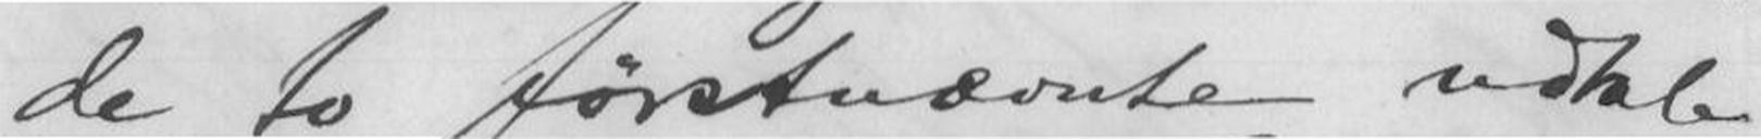de to førstnævnte udtale
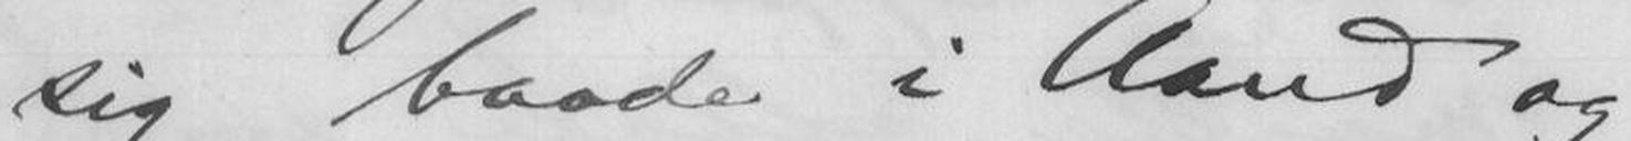sig baade i Aand og
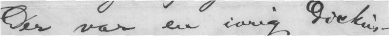Der var en ivrig Diskus-
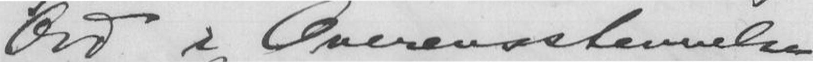Ord i Overensstemmelse
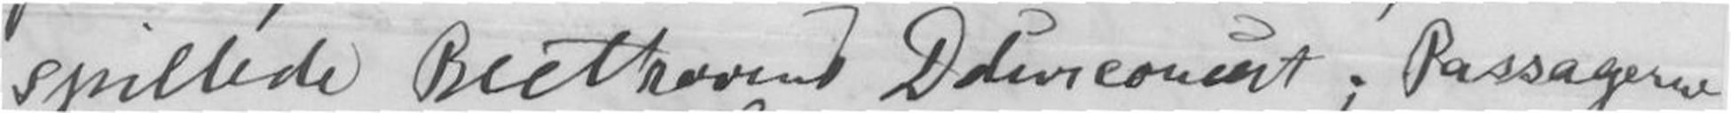spillede Beethovens Ddur concert; Passagerne,
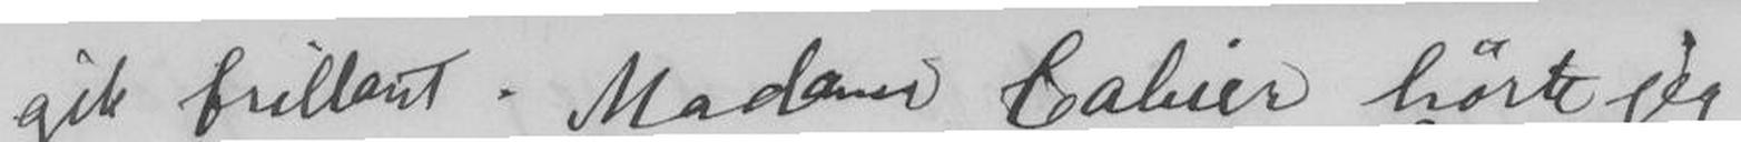gik brillant. Madam Cahier hørte jeg
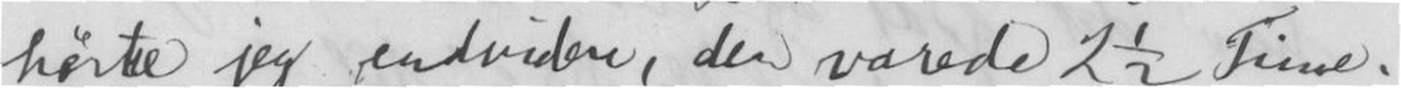hørte jeg endvidere, der varede 2½ Time.
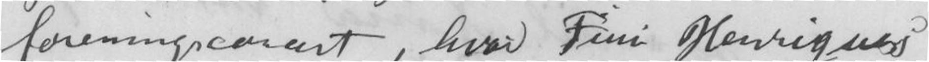foreningsconcert, hvori Fru Henriques
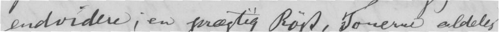endvidere; en prægtig Røst, Tonerne aldeles
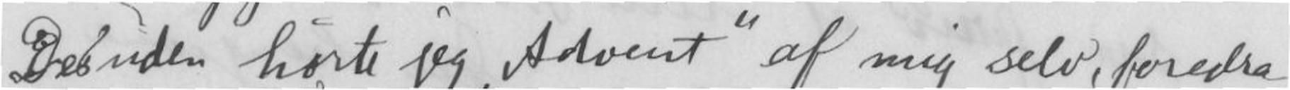Desuden hørte jeg Advent af mig selv, foredra-
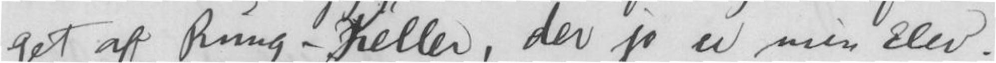get af Rung-Keller, der jo er min Elev.
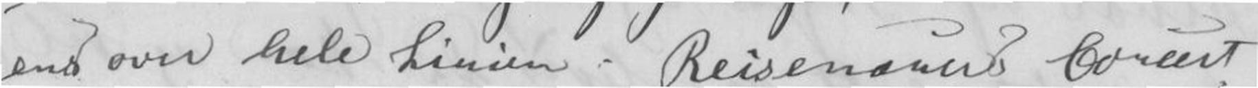ens over hele Linien. Reisenouers Concert
